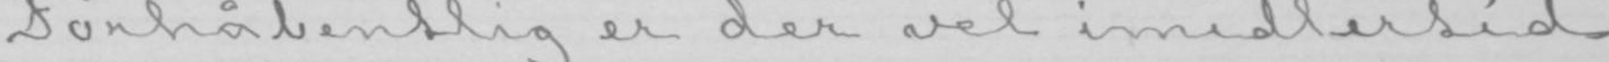Forhåbentlig er der vel imidlertid
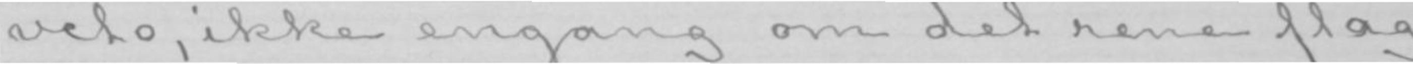veto, ikke engang om det rene flag,
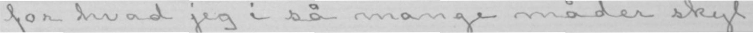for hvad jeg i så mange måder skyl-
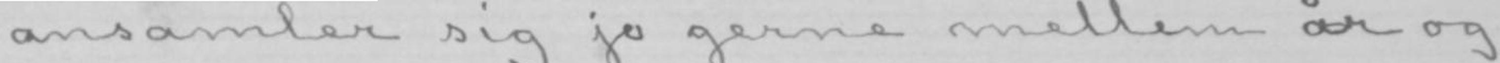ansamler sig jo gerne mellem år og
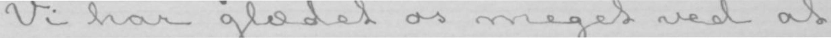Vi har glædet os meget ved at
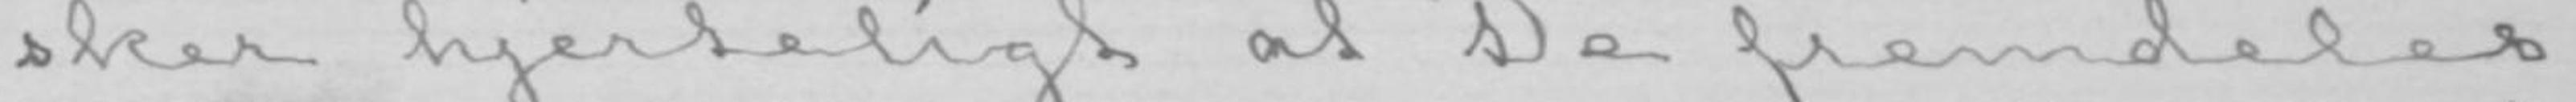sker hjerteligt at De fremdeles
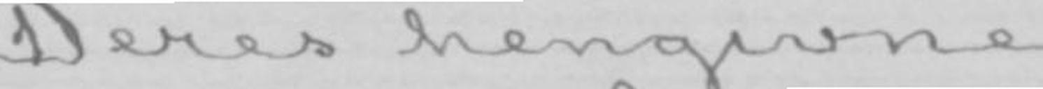Deres hengivne
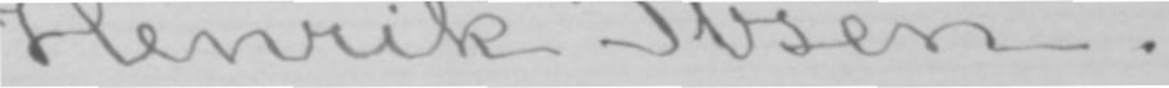Henrik Ibsen.
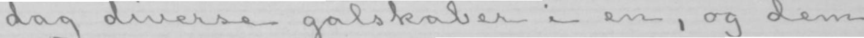dag diverse galskaber i en, og dem
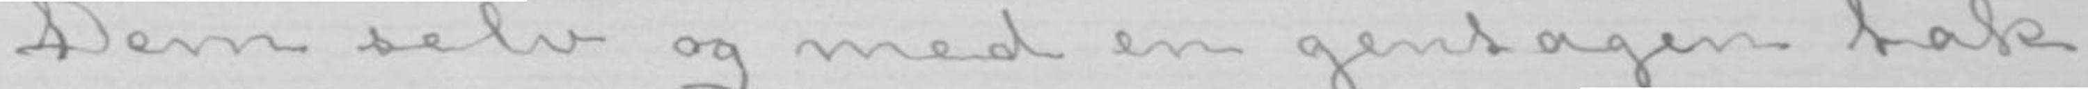Dem selv og med en gentagen tak
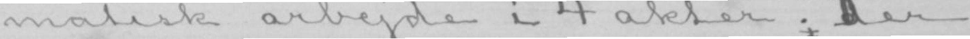matisk arbejde i 4 akter. Der
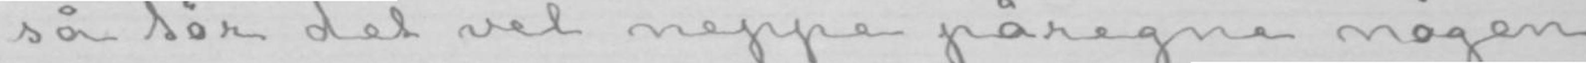så tør det vel neppe påregne nogen
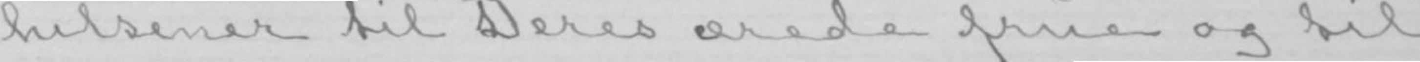hilsener til Deres ærede frue og til
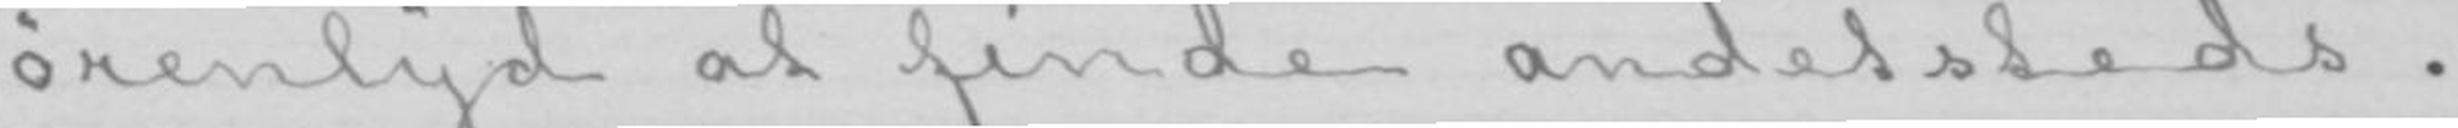ørenlyd at finde andetsteds.
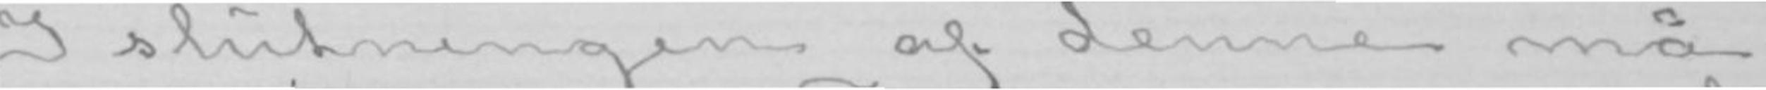I slutningen af denne må-
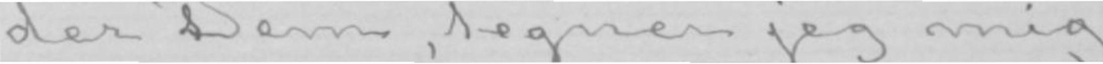der Dem, tegner jeg mig
